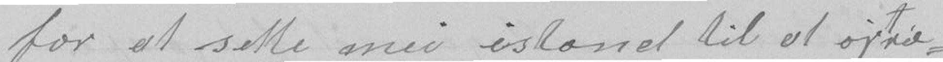for at sette mei istand til at optræ-
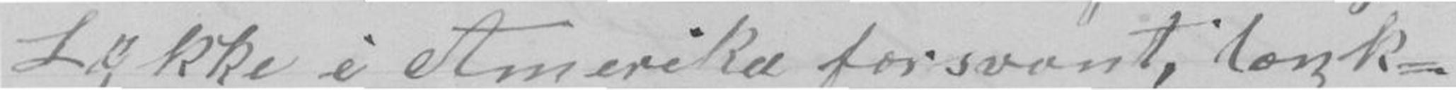Lykke i Amerika forsvant, tonk-
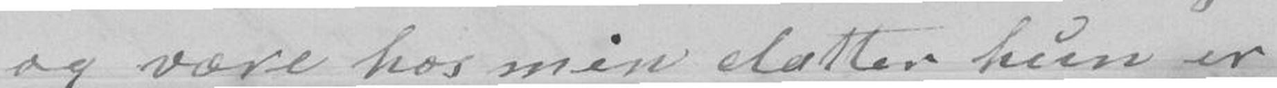og være hos min datter hun er
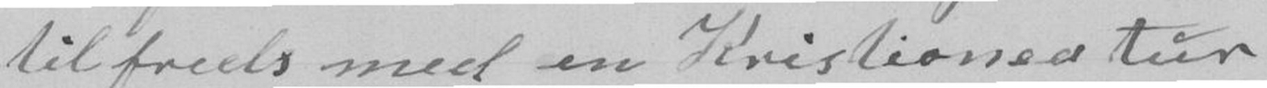tilfreds med en Kristianiatur
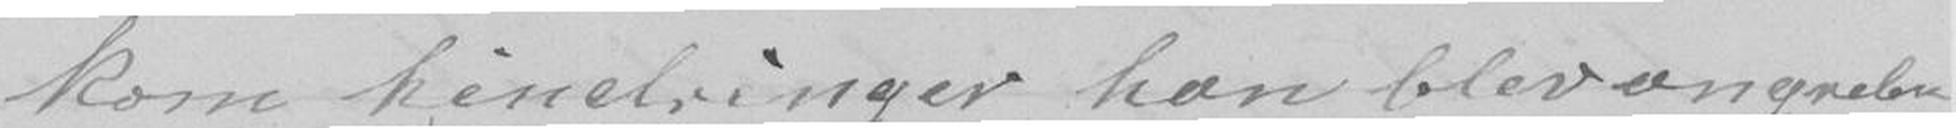kom hindringer han blev angreben
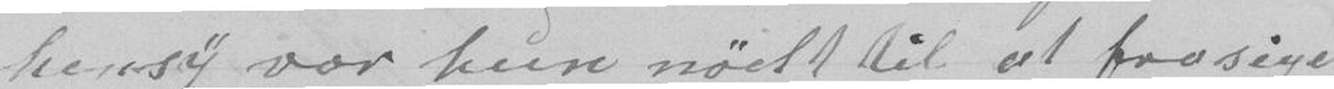hensy vor hun nødt til at frasige
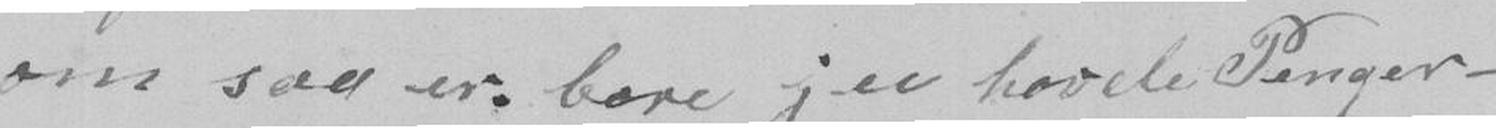om saa er. bare jei hovde Penger
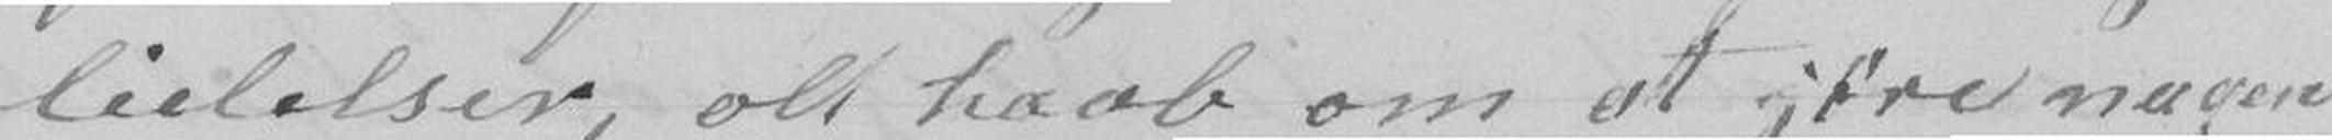lidelser, olt haab om at jøre nogen
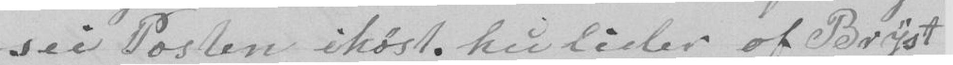sei Posten ihøst. hu lider of Bryst
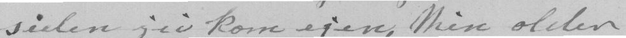siden jei kom ijen, Min older
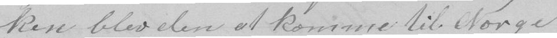ken blev den at komme til Norge
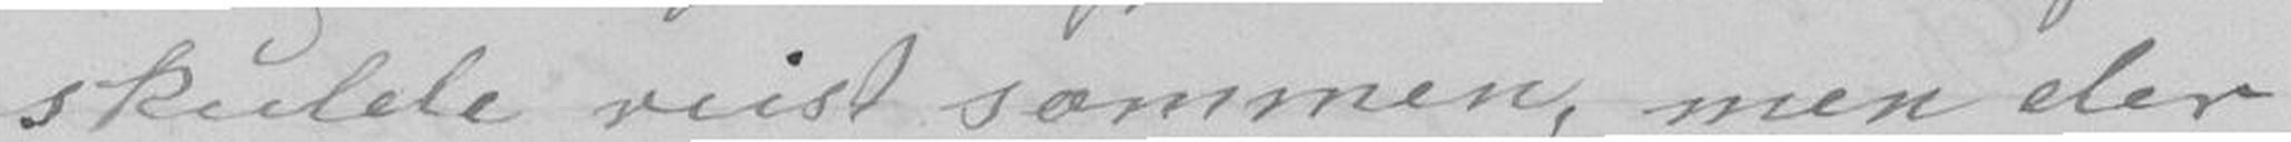skulde reist sammen, men der
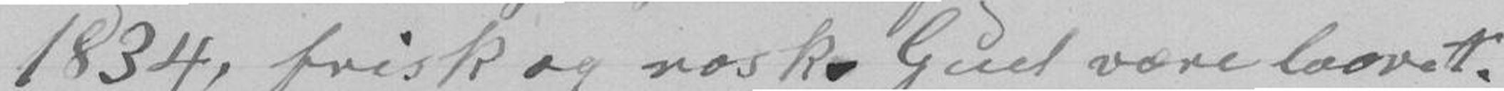1834, frisk og rosk. Gud være laavet.
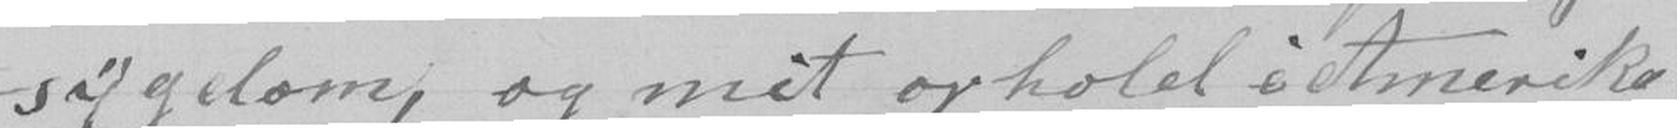sygdom og mit ophold i Amerika
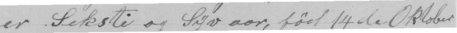er Seksti og Syv aar, fød 14de Oktober
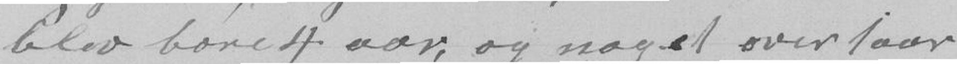blev bare 4 aar, og noget over 1 aar
